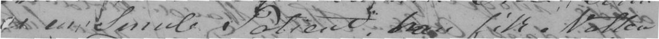er en Smule Patient, han fik Natten
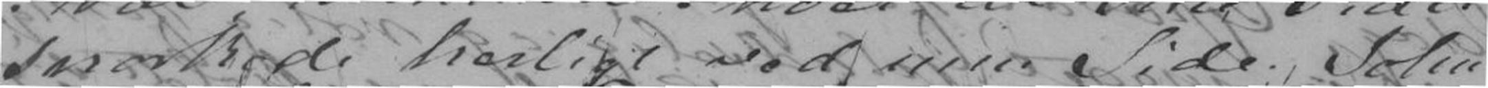snorkede herligt ved min Side. John
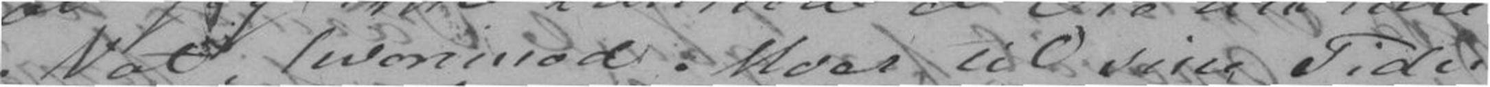Nat hvorimod Moer til sine Tider
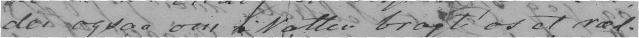der ogsaa om Natten bragte os et ræd-
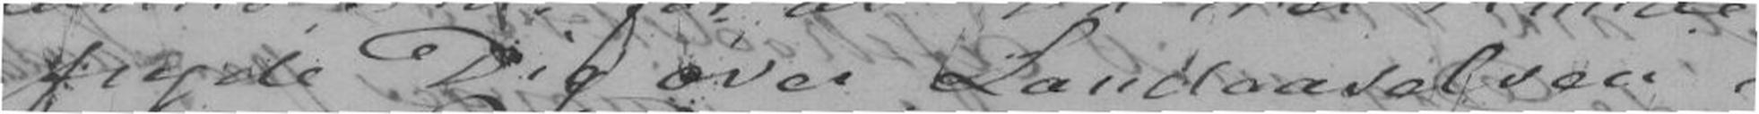fryde Dig over Landaaselven i
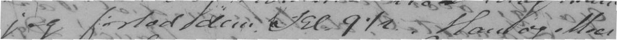jeg forlod dem Kl.9½. Han og Moer
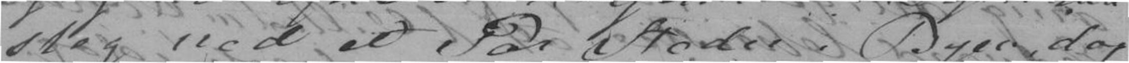slog ned et Par Steder i Byen, dog
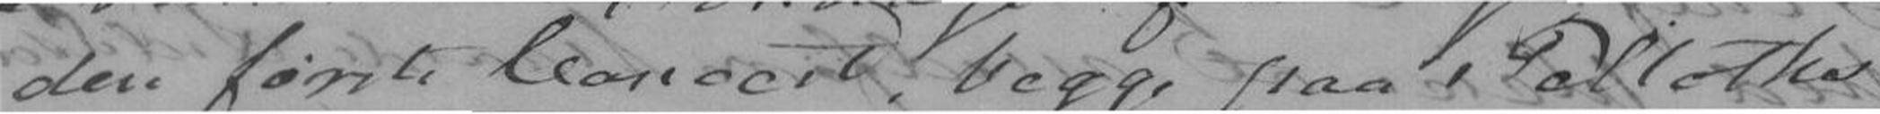den første Concert begge paa Polloths
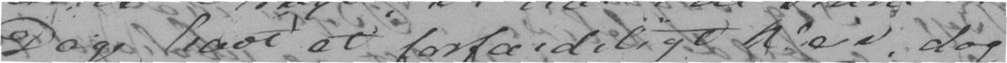Dage havt et forfærdeligt Veir, dog
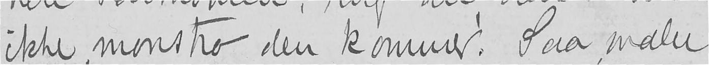ikke, monstro den kommer! Saa maler
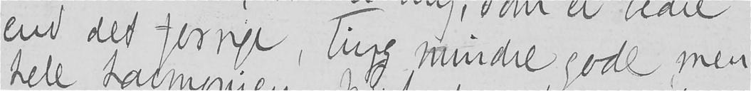end det forrige, ting mindre gode, men
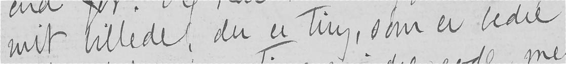mit billede, der er ting, som er bedre
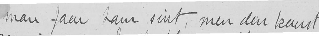man faar ham sint, men den kunst
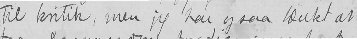til kritik, men jeg har ogsaa tænkt at
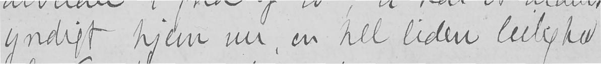yndigt hjem nu, en hel liden leilighed
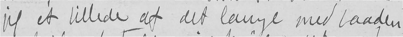jeg et billede af det lange med baaden
